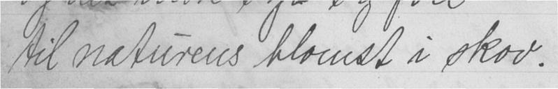til naturens blomst i skov.
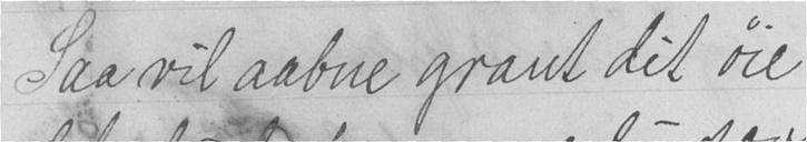Saa vil aabne grant dit øie
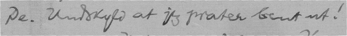De. Undskyld at jeg prater bent ut!
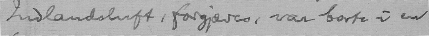Indlandsluft, forgjæves, var borte i en
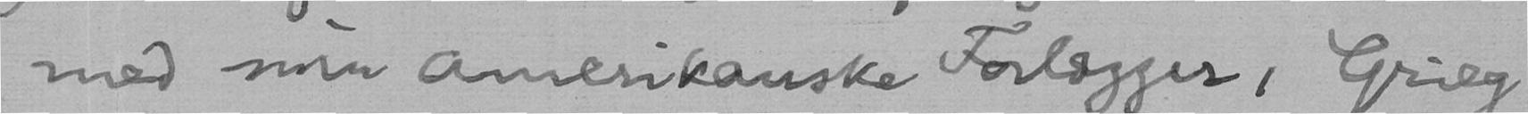med min amerikanske Forlægger, Grieg
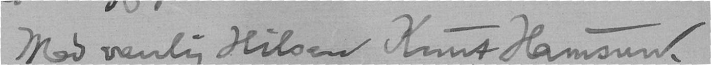Med venlig Hilsen Knut Hamsun.
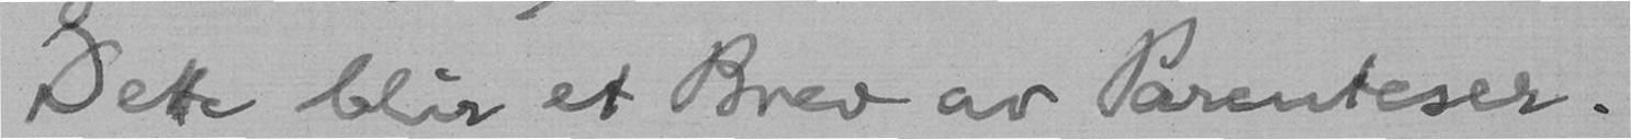Dette blir et Brev av Parenteser.
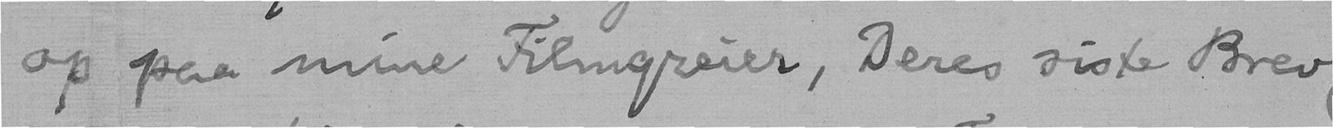op paa mine Filmgreier, Deres siste Brev
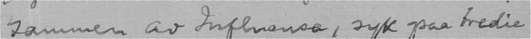sammen av Influensa, syk paa tredie
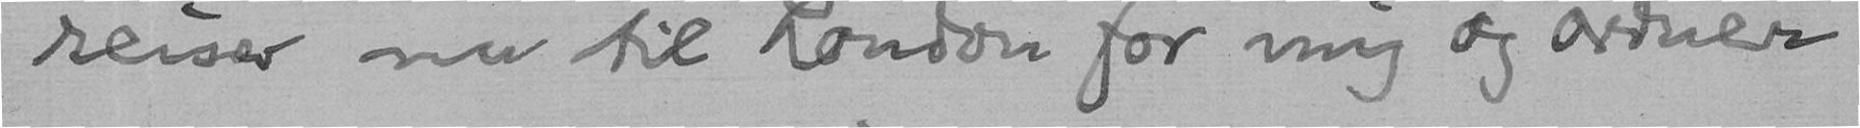reiser nu til London for mig og ordner
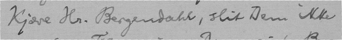Kjære Hr. Bergendahl, slit Dem ikke
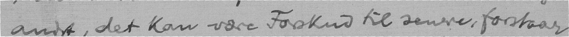andet, det kan være Forskud til senere, forstaar
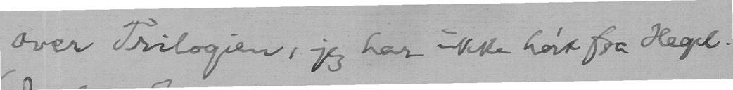over Trilogien, jeg har ikke hørt fra Hegel.
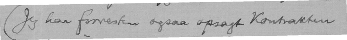(Jeg har forresten ogsaa opsagt Kontrakten
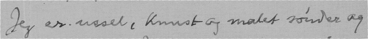Jeg er ussel, knust og malet sønder og
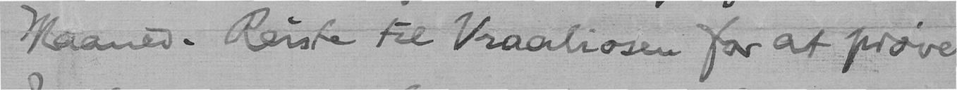Maaned. Reiste til Vraaliosen for at prøve
